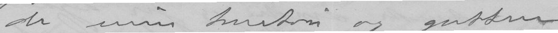de min hustru og gutten
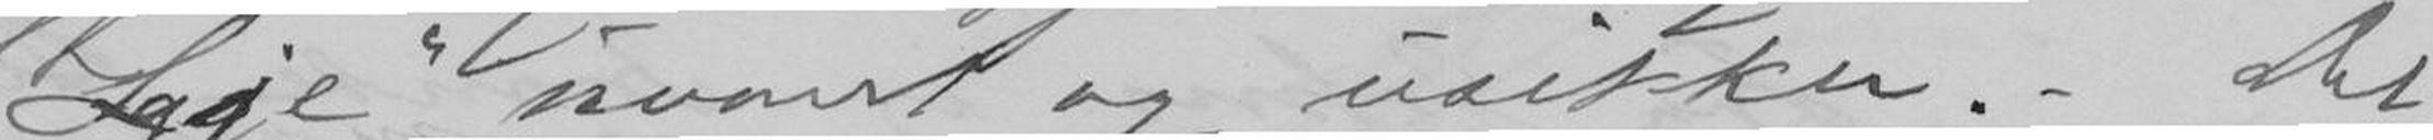"Leje" uvant og usikker. - Det
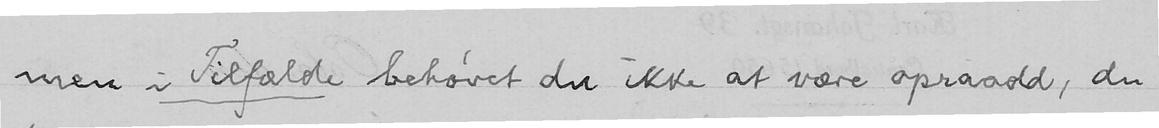men i Tilfælde behøvet du ikke at være opraadd, du
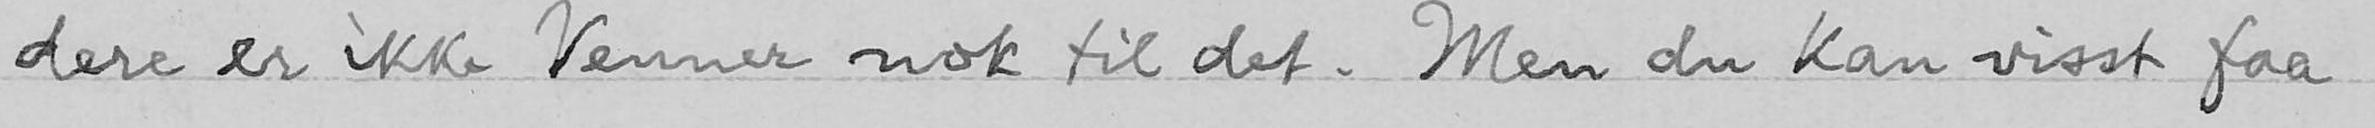dere er ikke Venner nok til det. Men du kan visst faa
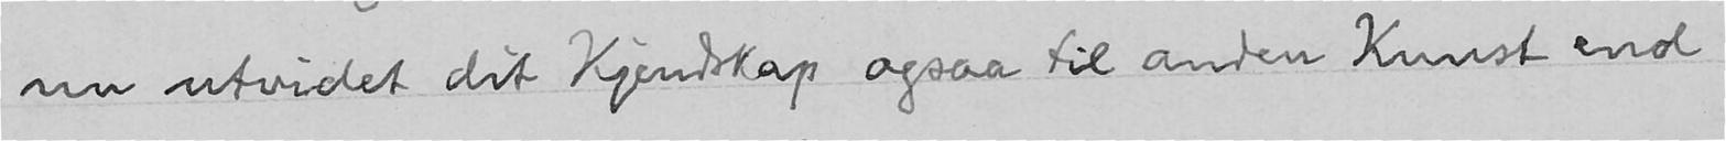nu utvidet dit Kjendskap ogsaa til anden Kunst end
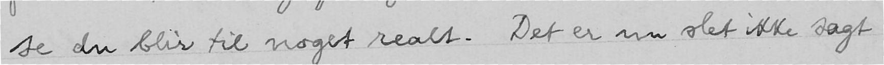se du blir til noget realt. Det er nu slet ikke sagt
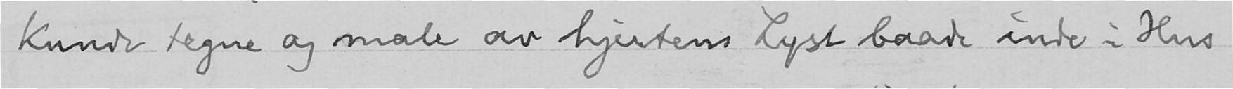kunde tegne og male av hjertens Lyst baade inde i Hus
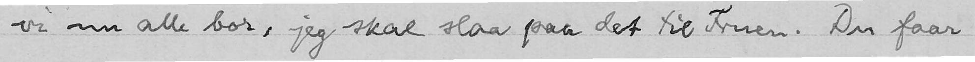vi nu alle bor, jeg skal slaa paa det til Fruen. Du faar
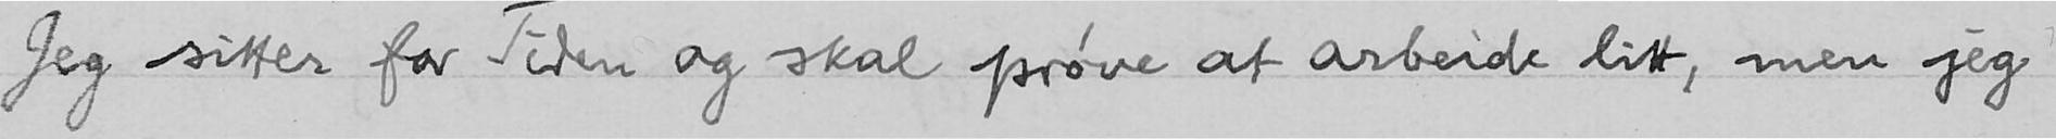Jeg sitter for Tiden og skal prøve at arbeide litt, men jeg
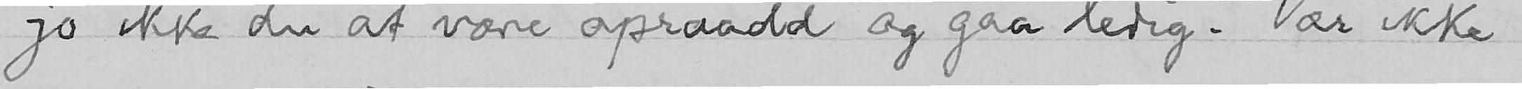jo ikke du at være opraadd og gaa ledig. Vær ikke
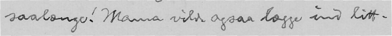saalænge! Mama vilde ogsaa lægge ind litt.
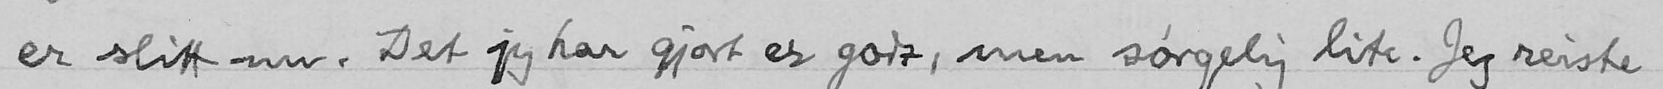er slitt nu. Det jeg har gjort er godt, men sørgelig lite. Jeg reiste
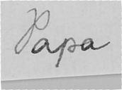Papa
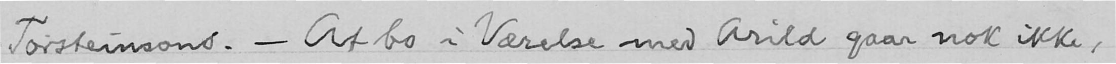Torsteinsons. - At bo i Værelse med Arild gaar nok ikke,
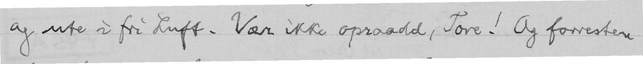og ute i fri Luft. Vær ikke opraadd, Tore! Og forresten
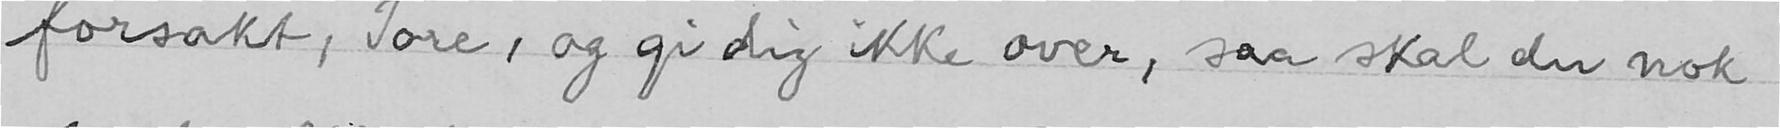forsakt, Tore, og gi dig ikke over, saa skal du nok
